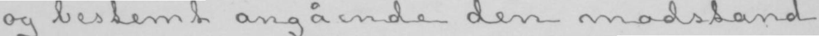og bestemt angående den modstand,
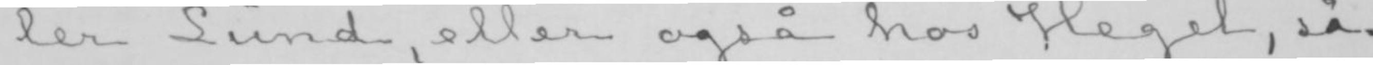ler Lund, eller også hos Hegel, så-
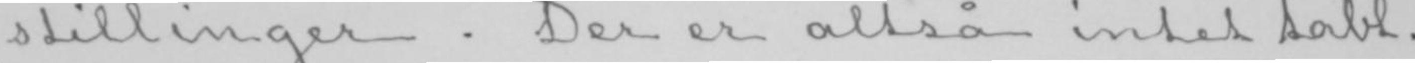stillinger. Der er altså intet tabt.
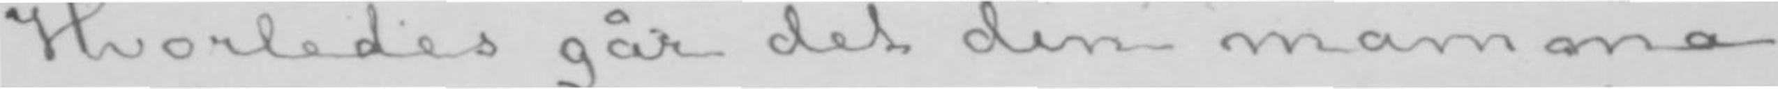Hvorledes går det din mamma
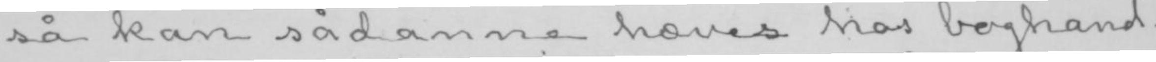så kan sådanne hæves hos boghand-
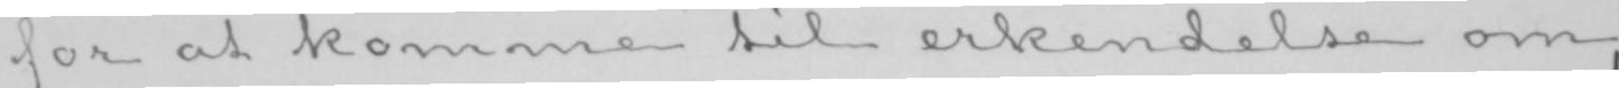for at komme til erkendelse om,
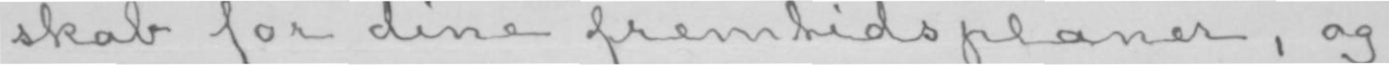skab for dine fremtidsplaner, og
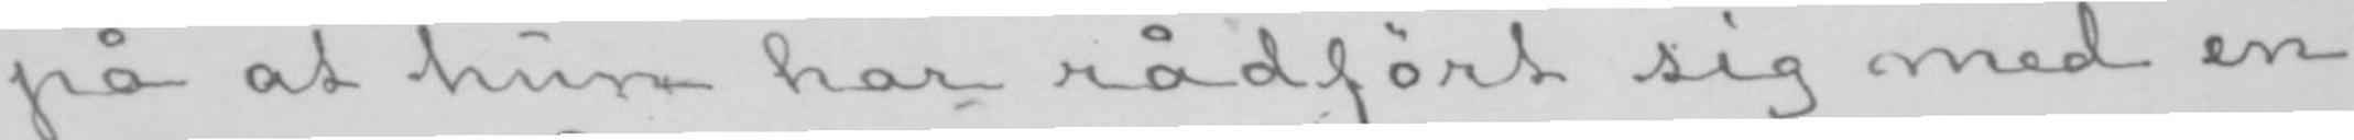på at hun har rådført sig med en
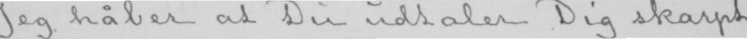Jeg håber at Du udtaler Dig skarpt
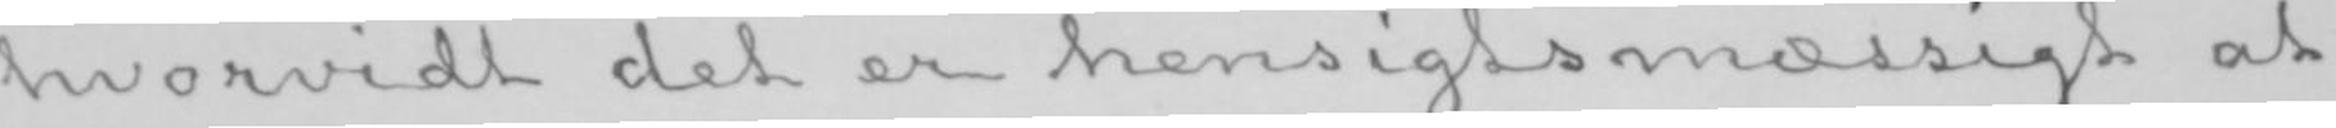hvorvidt det er hensigtsmæssigt at
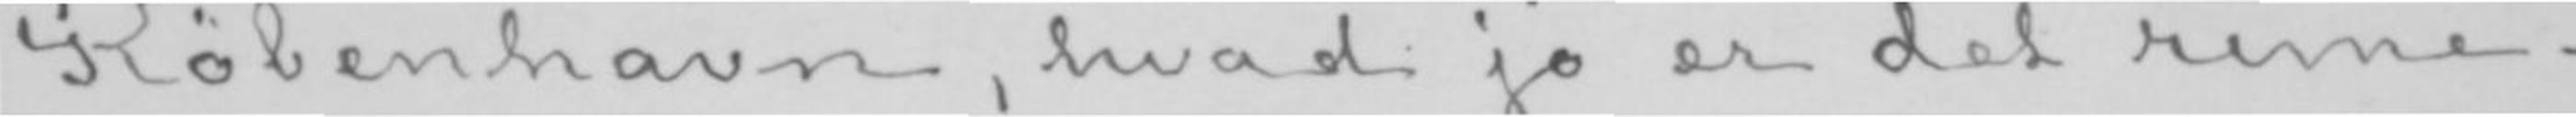København, hvad jo er det rime-
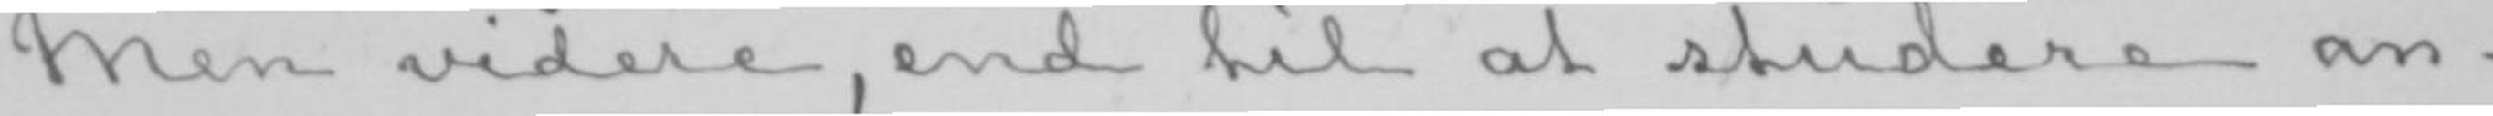Men videre, end til at studere an-
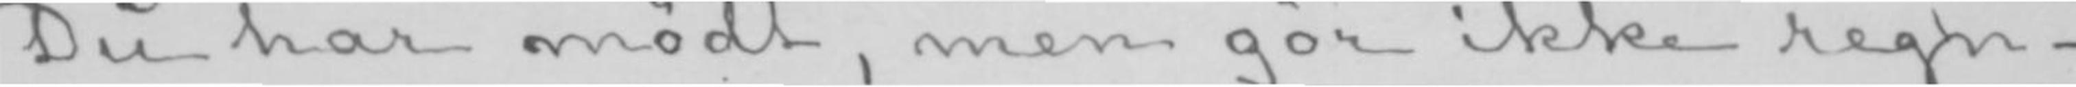Du har mødt, men gør ikke regn-
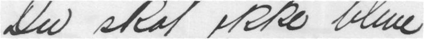Du skal ikke blive
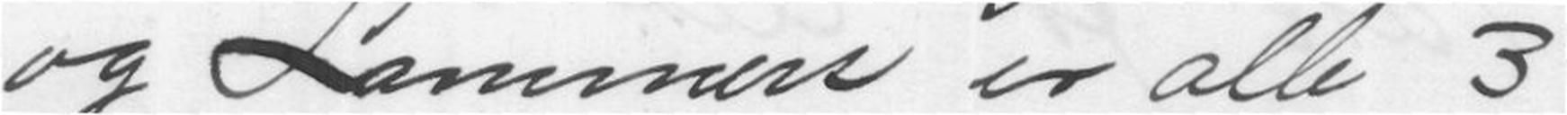og Lammers er jo alle 3
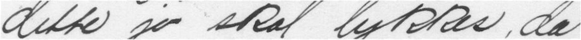dette jo skal lykkes, da
